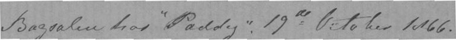Bogsalen hos "Paddy". 19de October 1866.
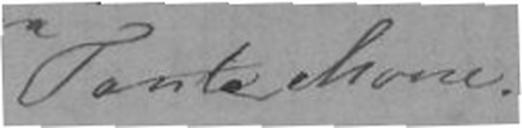Tante Mone
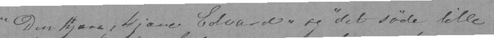"Du kjære, kjære Edvard" og "det søde lille
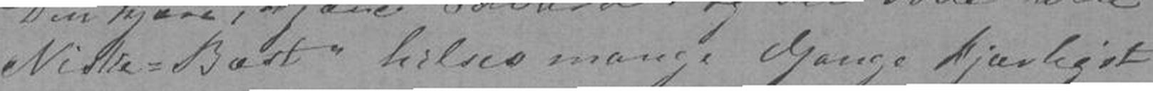Niske-Bast" hilses mange Gange kjærligst
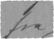fra
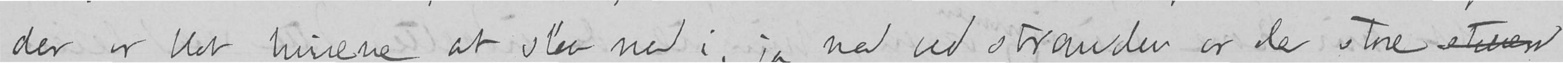der er blot husene at slaa ned i, ja ned ved stranden er de store stenen
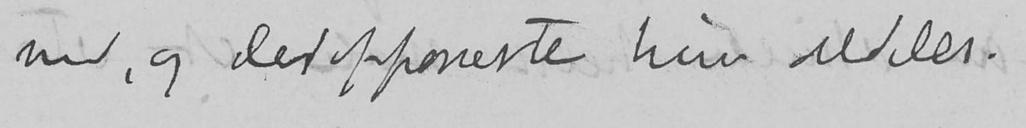med, og der opponerte hun aldeles.
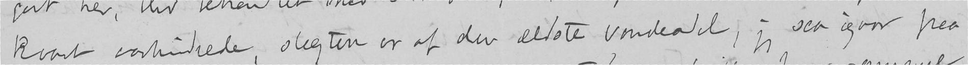kvart aarhundrede, slægten er af den ældste bondeadel, jeg saa igaar paa
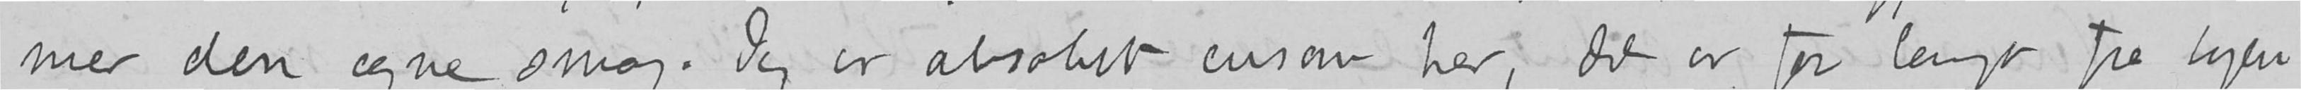mer den egne smag. Jeg er absolut ensom her, det er for langt fra byen
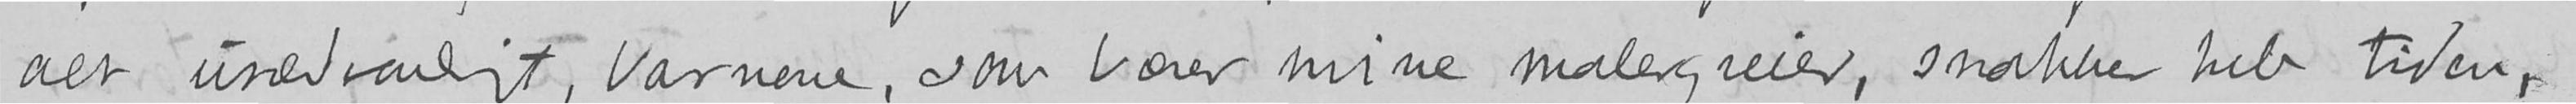alt usædvanligt, barnene, som bærer mine malergreier, snakker hele tiden,
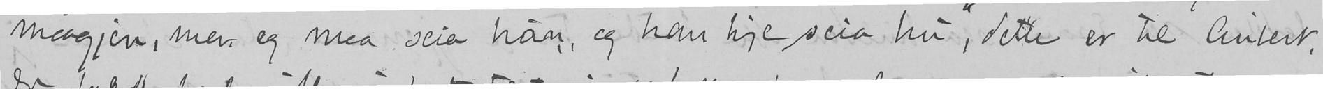maagjen, men eg maa seia hun, eg kan kje seia hu", dette er til Aubert,
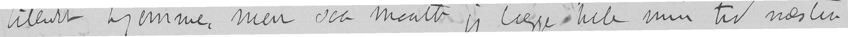billedet hjemme, men saa maatte jeg lægge hele min tid næsten
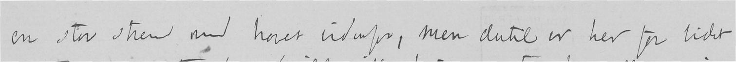en stor strand med havet udenfor, men dertil er her for lidet
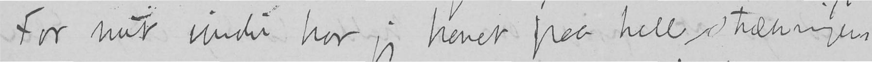For mit vindu har jeg hevet paa hell strækningen
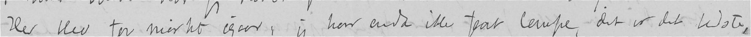Her blev for mørkt igaar, jeg har enda ikke faat lampe, det er det bedste,
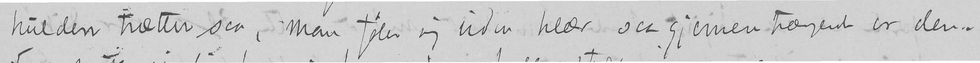kulden trækker saa, man føler sig uden klær saa gjennemtrængende er den.
